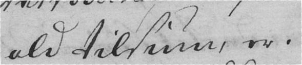ald tilsiun, er.
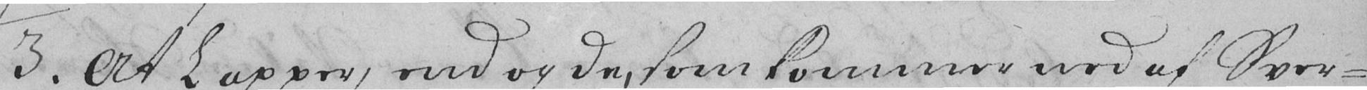3. At Lapper, end og de, som kommer ned af Sver-
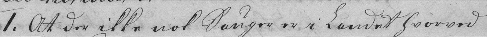1. At der ikke nok Sauger er i Landet hvorved
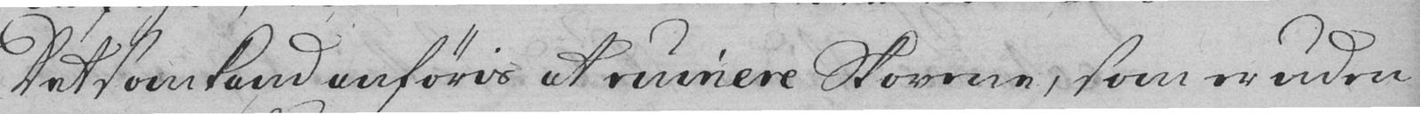Det som kand anføris at ruinere Skovene, som er uden
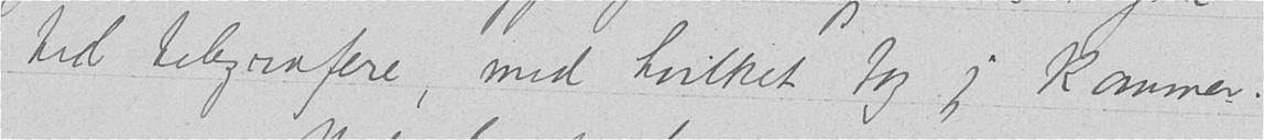tid telegrafere, med hvilket tog jeg kommer.
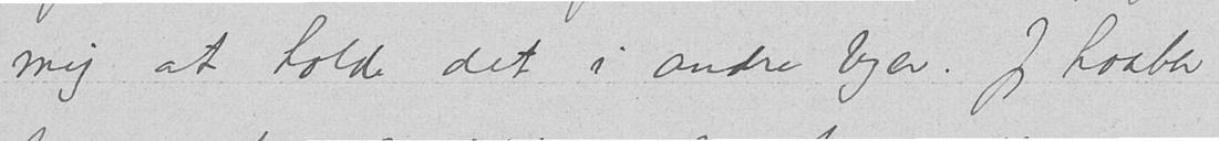mig at holde det i andre byer. Jeg haaber
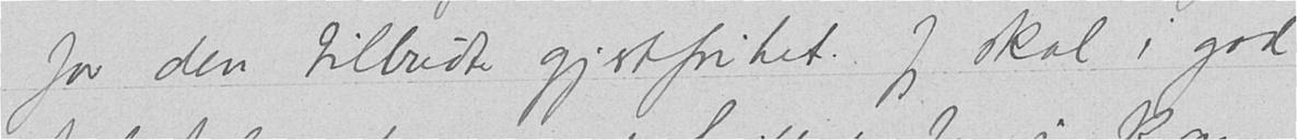for den tilbudte gjestfrihet. Jeg skal i god
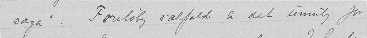saga". Foreløbig ialfald er det umulig for
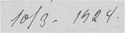10/3-1924.
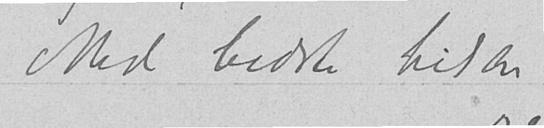Med bedste hilsen
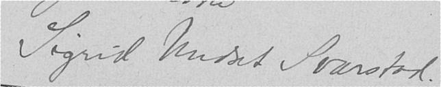Sigrid Undset Svarstad.
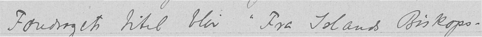Foredragets titel blir "Fra Islands Biskops-
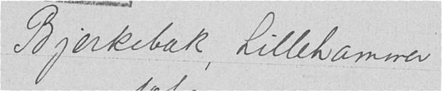Bjerkebæk, Lillehammer
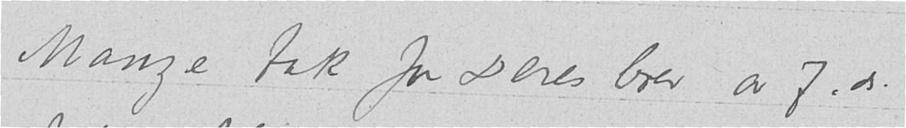Mange tak for Deres brev av 7. ds.
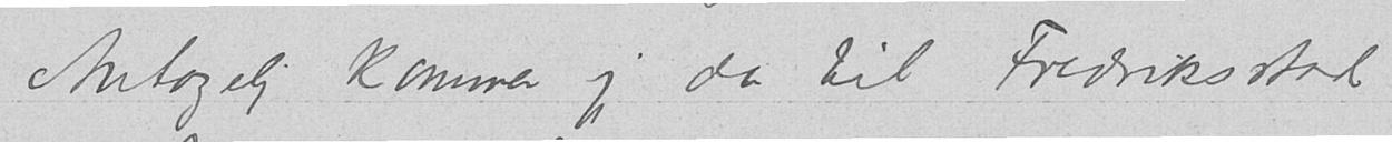Antagelig kommer jeg da til Fredriksstad
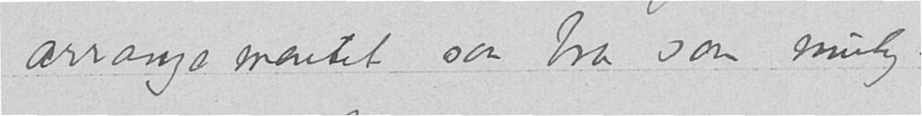arrangementet saa bra som mulig.
